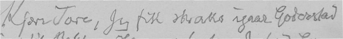Kjære Tore, Jeg fik straks igaar Goderstad
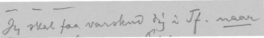Jeg skal faa varskud dig i Tf. naar
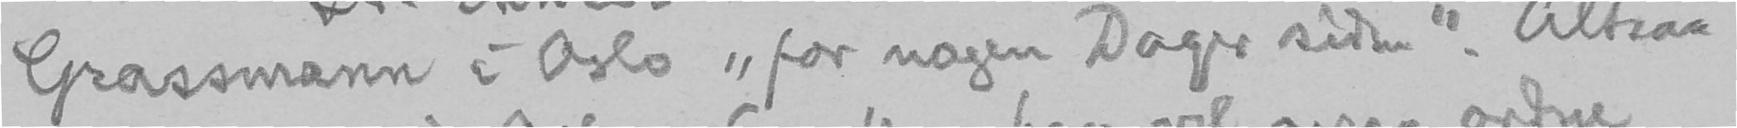Grassmann i Oslo "for nogen Dager siden". Altsaa
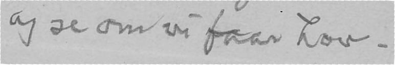og se om vi faar Lov.
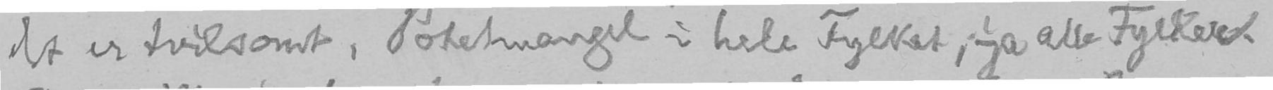det er tvilsomt, Potetmangel i hele Fylket, ja alle Fylker.
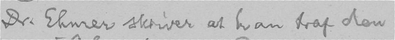Dr. Ehmer skriver at han traf den
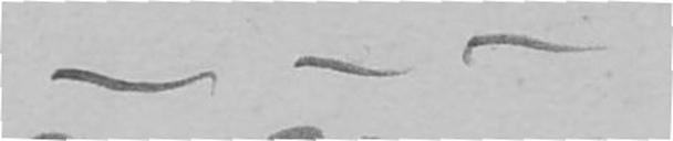- - -
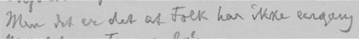Men det er det at Folk har ikke engang
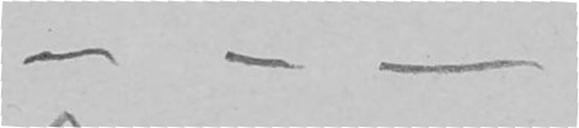- - -
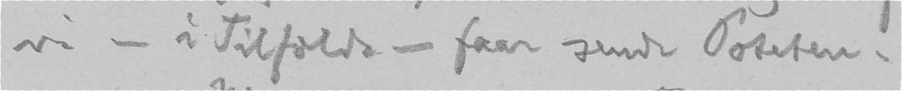vi - i Tilfælde - faar sende Poteten.
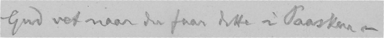Gud vet naar du faar dette i Paasken -
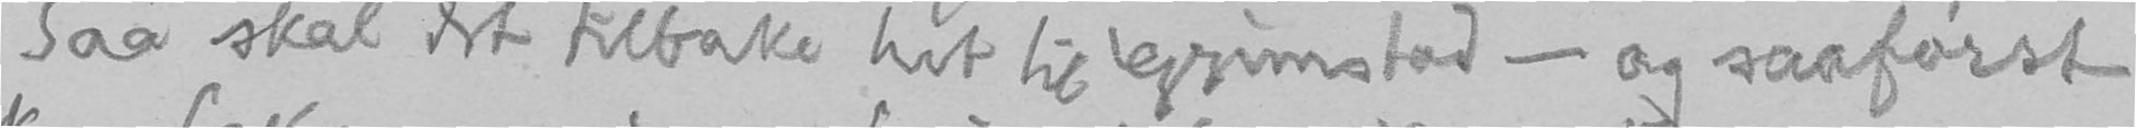Saa skal det tilbake hit til Grimstad - og saaførst
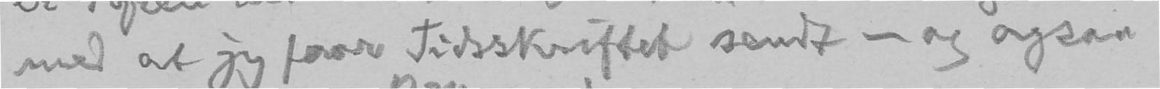med at jeg faar Tidsskriftet sendt - og ogsaa
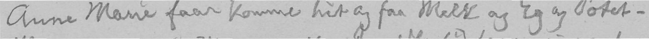Anne Marie faar komme hit og faa Melk og Eg og Potet.
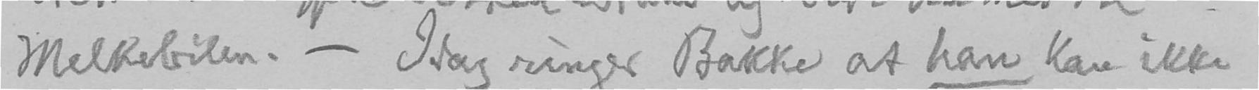Melkebilen. - Idag ringer Bakke at han kan ikke
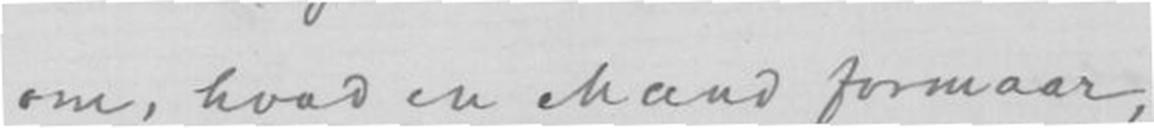om, hvad en Mand formaar,
